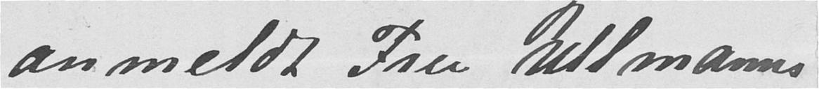anmeldt Fru Bullmanns
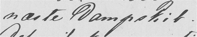næste Dampskib.
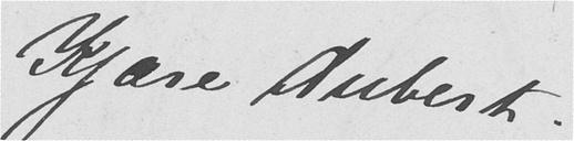Kjære Aubert.
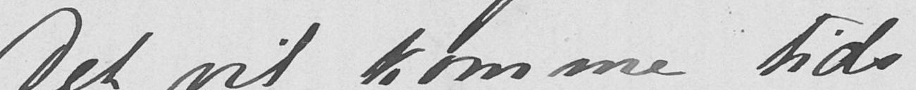Det vil komme tids-
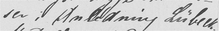ser i Anledning Lübeck.
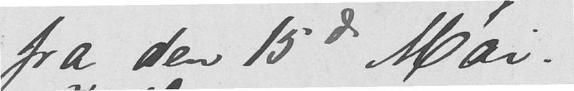fra den 15de Mai.
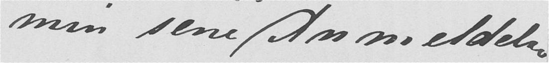min sene Anmeldelse
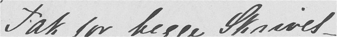Tak for begge Skrivel-
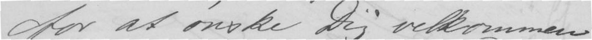for at ønske Dig velkommen
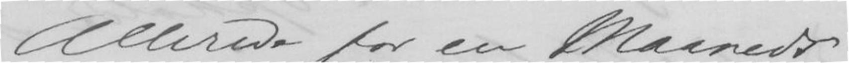Allerede for en Maaneds
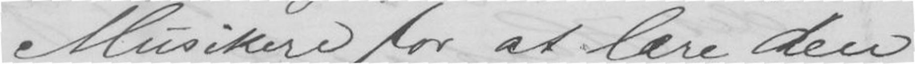Musikere for at lære dem
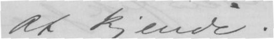at kjende.
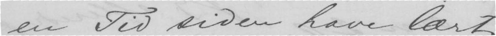en Tid siden have lært
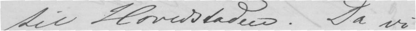til Hovedstaden. Da vi
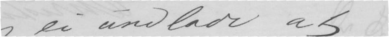jeg ei undlade at
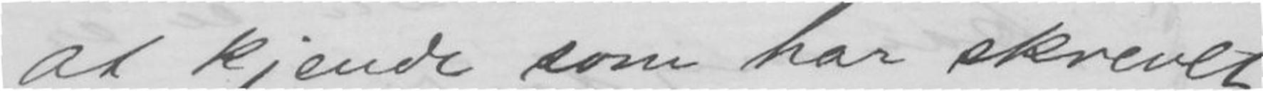at kjende som har skrevet
